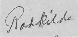Rødkilde
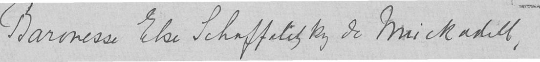Baronesse Else Schaffalitzky de Muckadell,
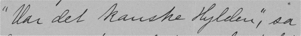"Var det kanske Hylden," sa
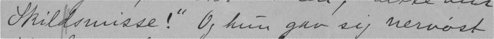Skildsmisse!" Og hun gav sig nervøst
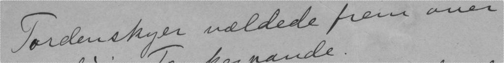Tordenskyer vældede frem over
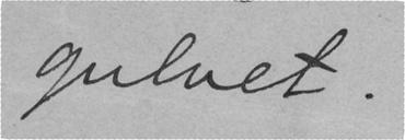gulvet
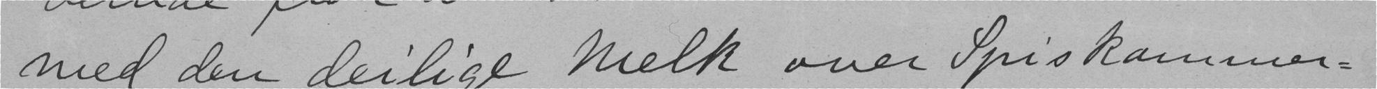med den deilige Melk over Spiskammer-
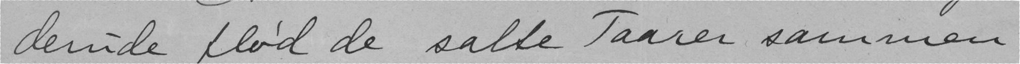derude flød de satte Taarer sammen
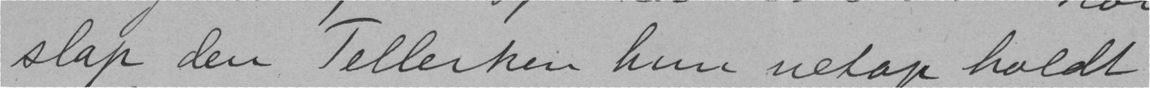slap den Tellerken hun netop holdt
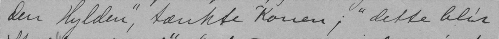den Hylden," tænkte Konen; " dette blir
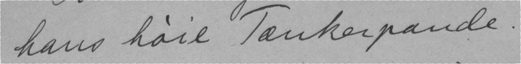hans høie Tænkerpande.
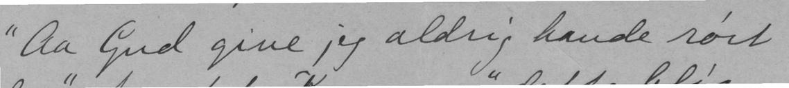"Aa Gud give jeg aldrig havde rørt
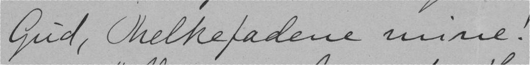Gud, Melkefadene mine!"
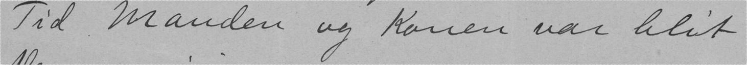Tid Manden og Konen var blit
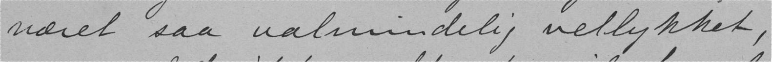været saa ualmindelig vellykket,
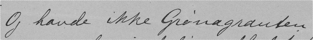Og havde ikke Grønagrauten
